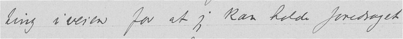ting i veien for at jeg kan holde foredraget
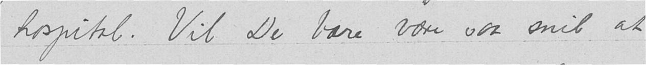hospital. Vil De bare være saa snil at
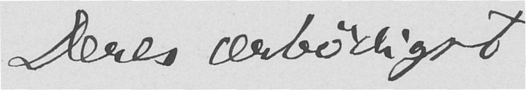Deres ærbødigst
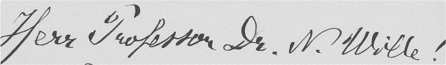Herr Professor Dr. N. Wille!
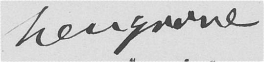hengivne
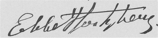Ebbe Hertzberg
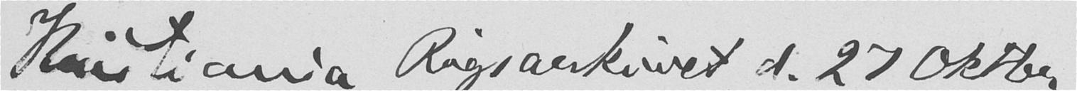Kristiania, Rigsarkivet, d. 27 Oktbr.
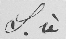S. u.
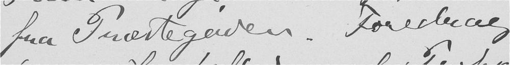fra Præstegaden. Foredrag
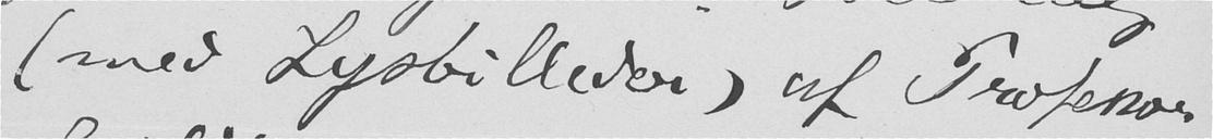(med Lysbilleder) af Professor
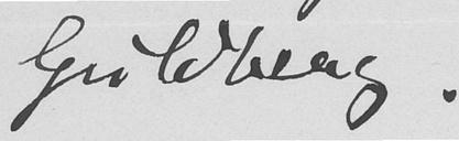Guldberg.
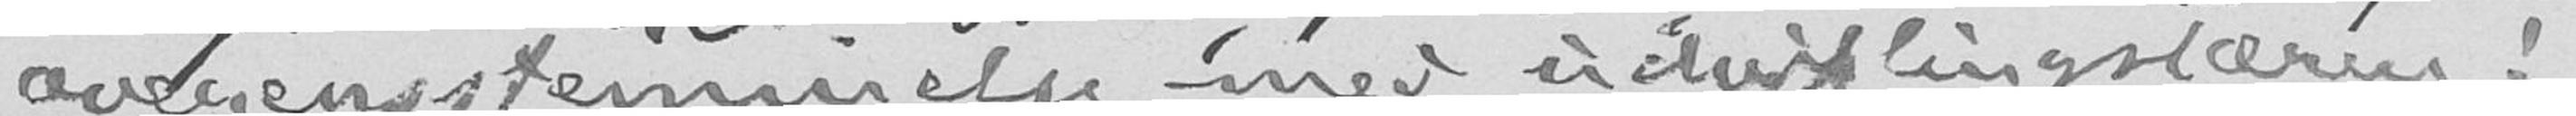overensstemmelse med udviklingslæren!
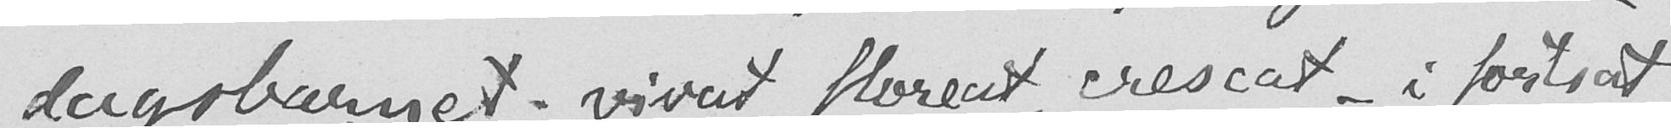dagsbarnet: vivat, floreat, crescat - i fortsat
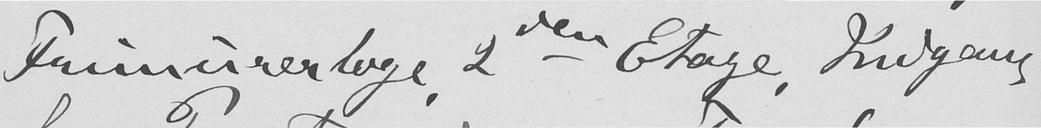Frimurerloge, 2den Etage, Indgang
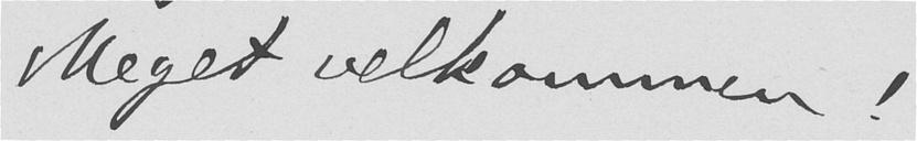Meget velkommen!
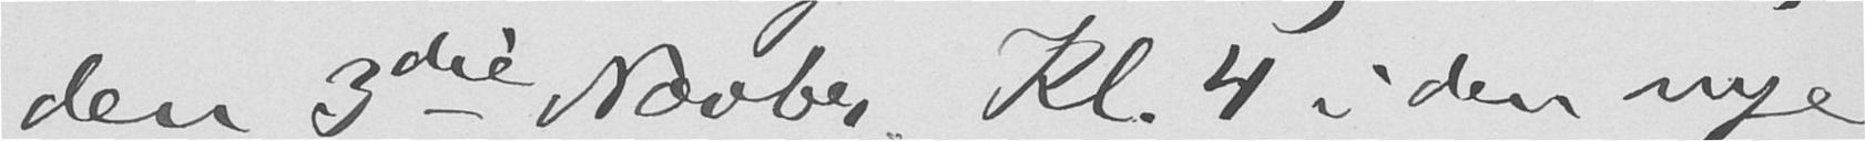den 3die Novbr. kl. 4 i den nye
